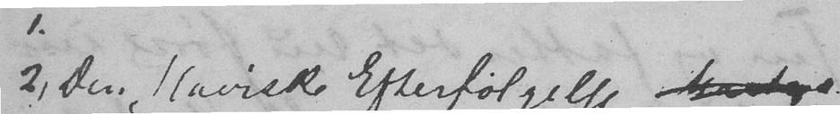2, Den slaviske Efterfølgelse
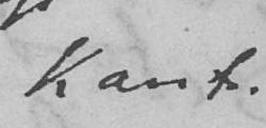Kant.
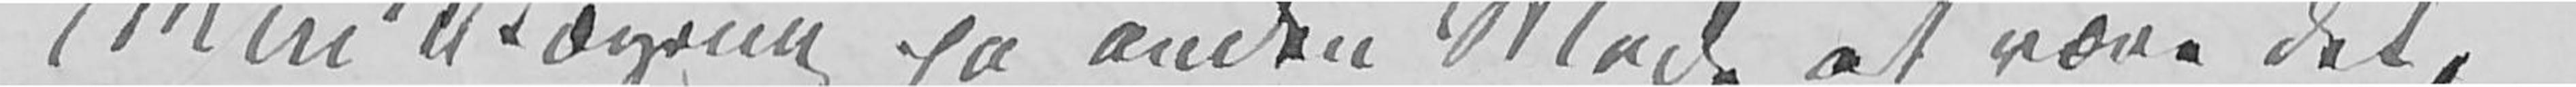Min Vægring på anden Måde at være det,
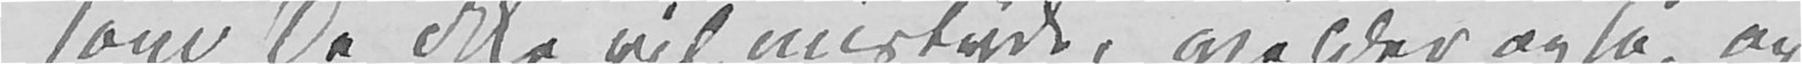som De ikke vil mistyde, gjelder også, og
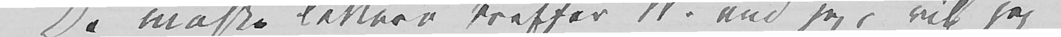De måske lettere treffer W. end jeg, vil jeg
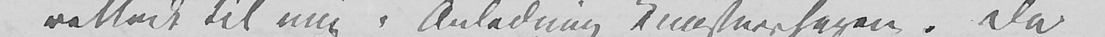rettede til mig i Anledning Kunstnersagen. Da
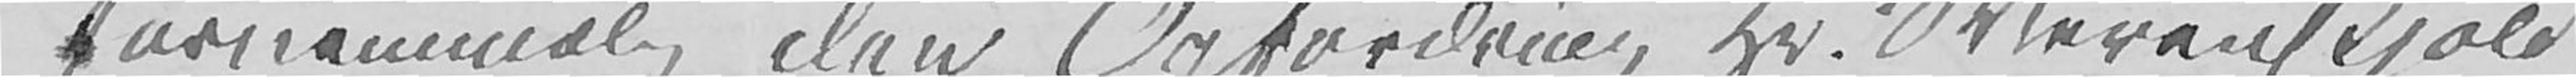fornemmelig den Opfordring Hr. Werenskjold
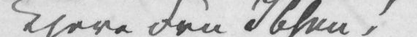Kjere Fru Ibsen!
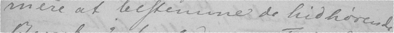mere at bestemme de hidhørende
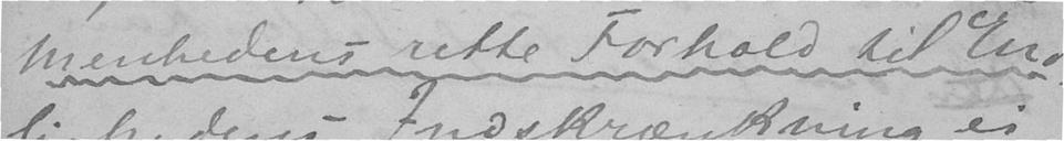menhedens rette Forhold til Ende-
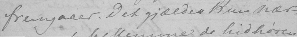fremgaaer. Det gjælder kun nær-
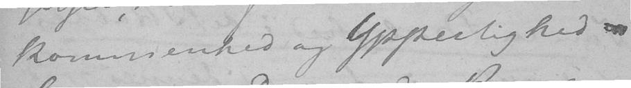kommenhed og Ypperlighed
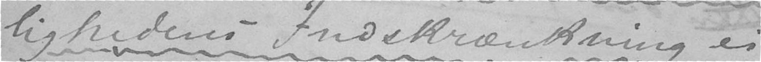lighedens Indskrænkning ei
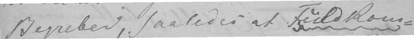Begreber, saaledes at Fuldkom-
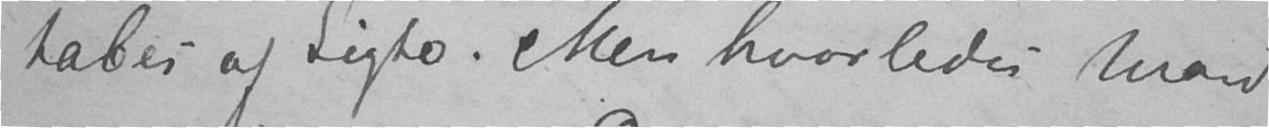tabes af Sigte. Men hvorledes man
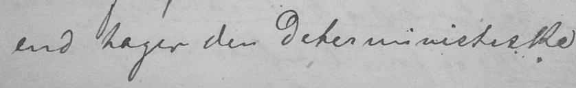end tager den deterministiske
